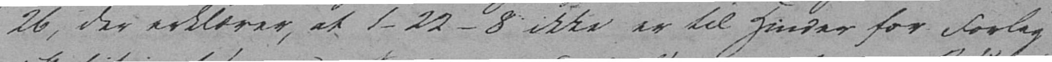26, der erklærer, at 1-22-8 ikke er til Hinder for Forlag
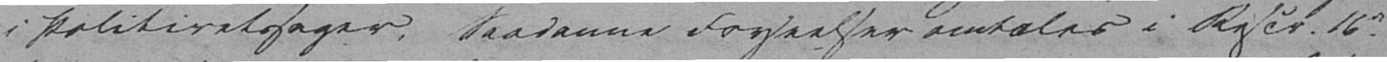i Politiretssager, saadanne Forseelser omtales i Rescr. 16d
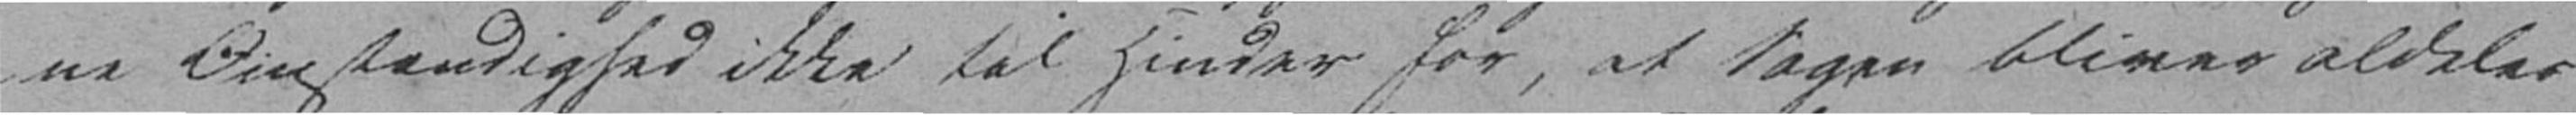ne Omstændighed ikke til Hinder for, at Sagen bliver aldeles
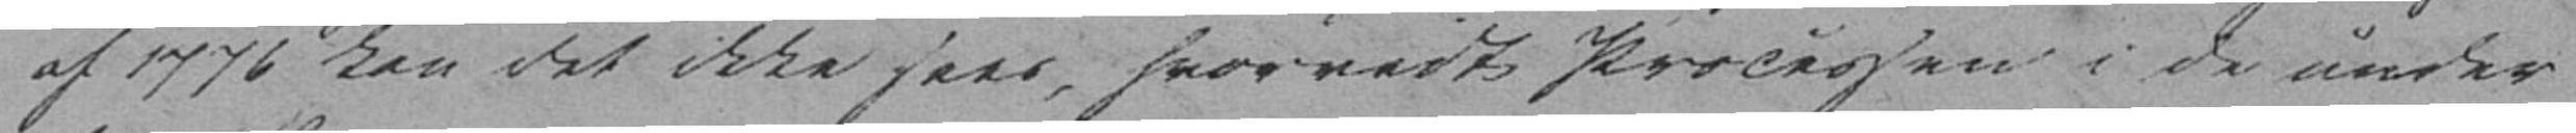af 1776 kan det ikke sees, hvorvidt Processen i de under
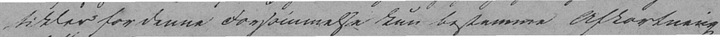tikler for denne Forsømmelse kun bestemme Afkortning
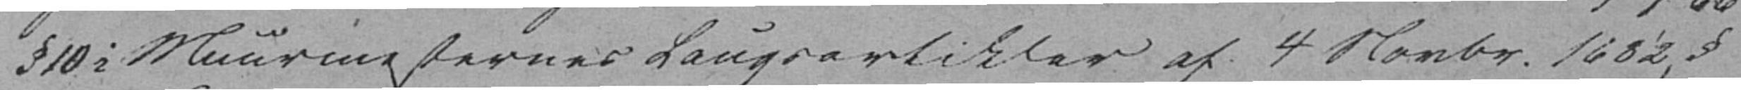§ 10 Muurmesternes Laugsartikler af 4 Novbr. 1682, §
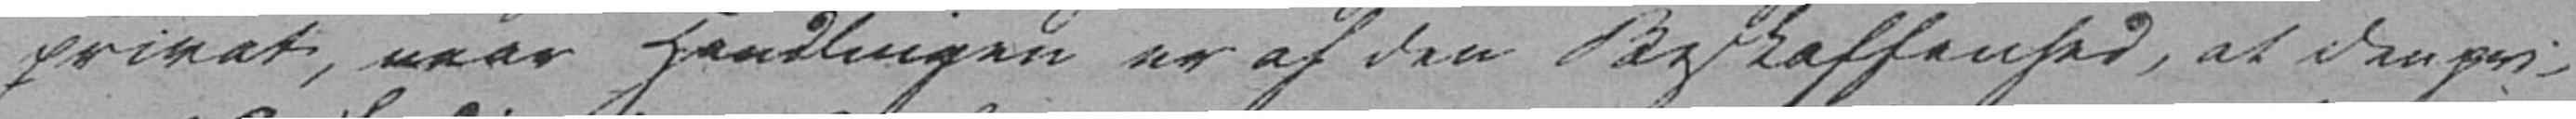privat, naar Handlingen er af den Beskaffenhed, at den pri-
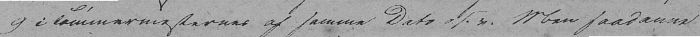9 i Tømmermesternes af samme Dato o. s. v. Men saadanne
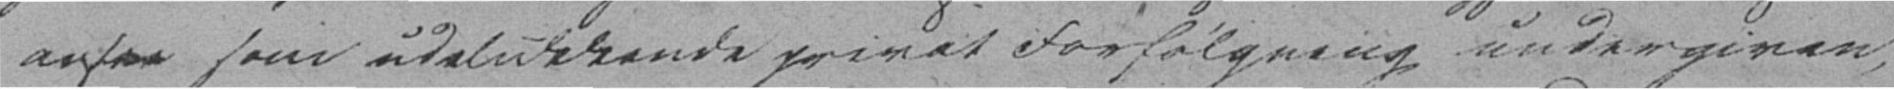ansee som udelukkende privat Forfølgning undergiven,
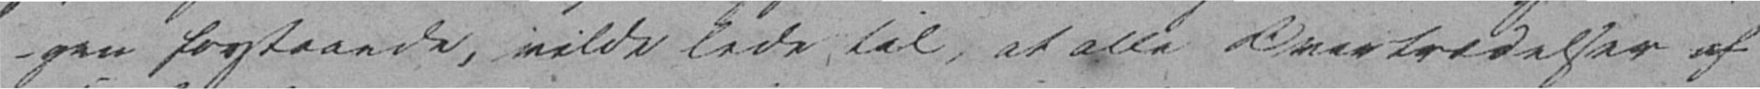gen forstaaende, vilde lede til, at alle Overtrædelser af
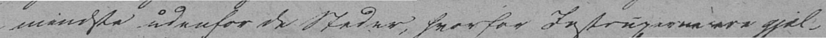mindste udenfor de Steder, hvorfor Instruxerne gjæl-
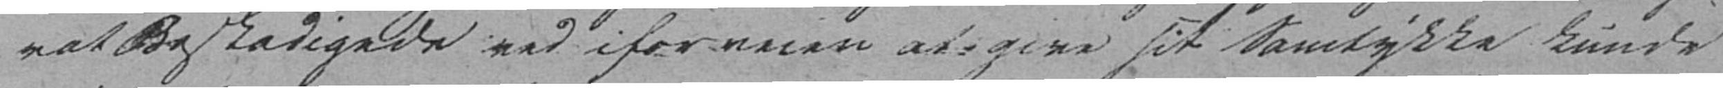vat Beskadigede ved iforveien at give sit Samtykke kunde
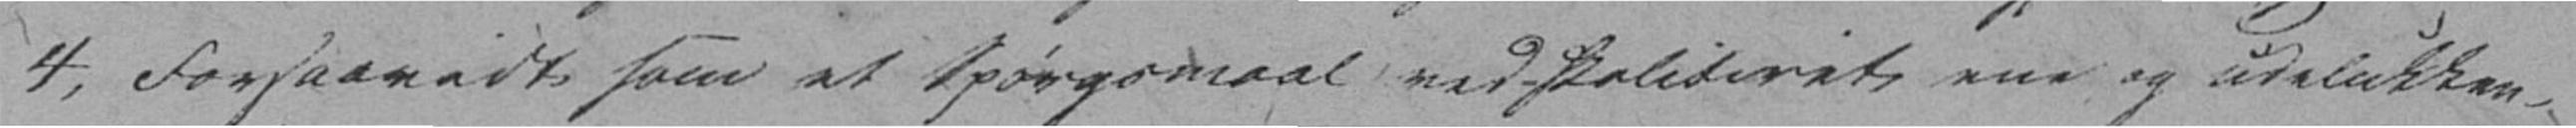4, Forsaavidt som et Spørgsmaal ved Politiret ene og udelukken-
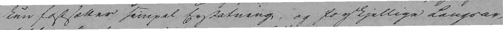kun fastsætter simpel Erstatning, og forskjellige Laugsar-
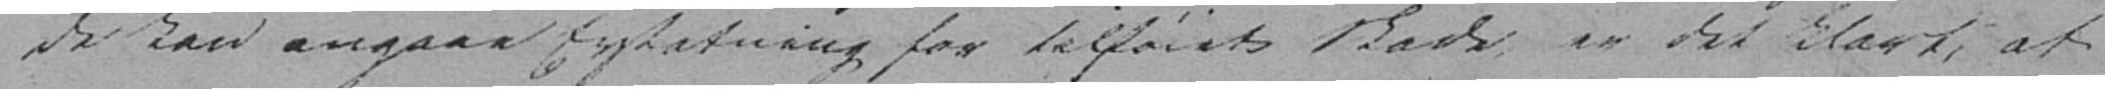de kan angaae Erstatning for tilføiet Skade, er det klart, at
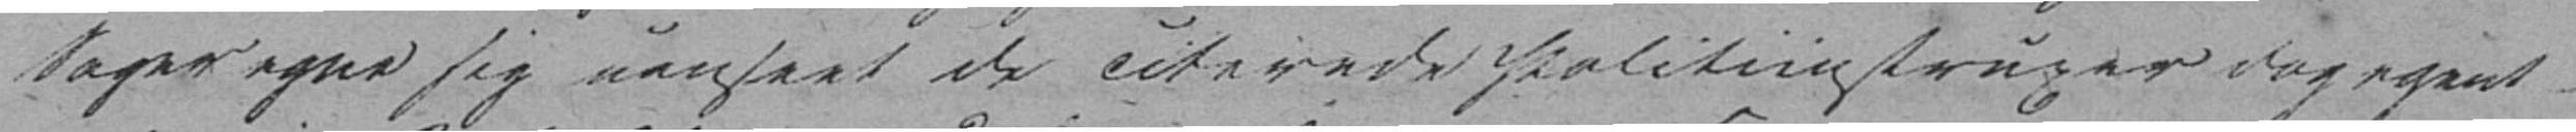Sager egne sig uanseet de citerede Politiinstruxer dog egent-
